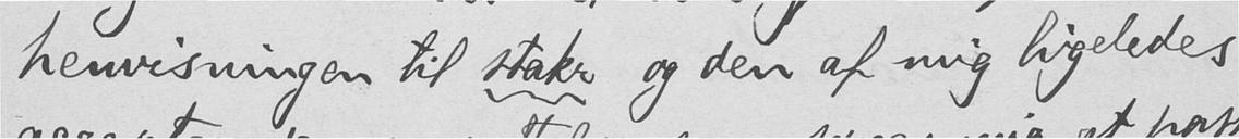henvisningen til stakr, og den af mig ligeledes
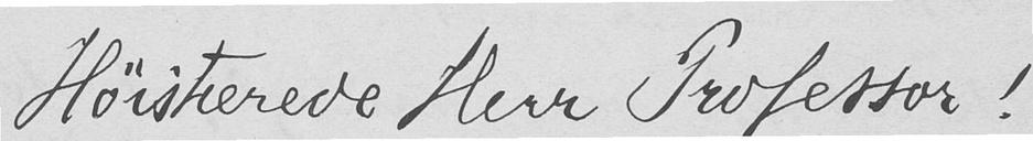Høistærede Herr Professor!
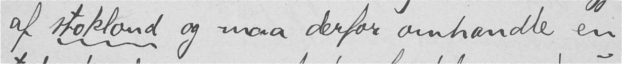af stoklond og maa derfor omhandle en
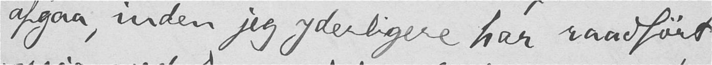afgaa, inden jeg yderligere har raadført
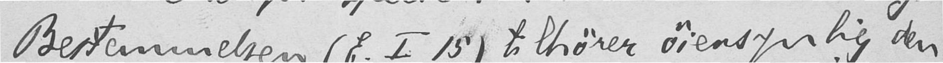Bestemmelsen (E. I 15) tilhører øiensynlig den
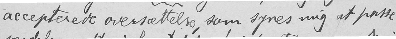accepterede oversættelse, som synes mig at passe
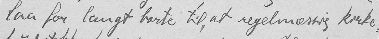laa for langt borte til, at regelmæssig kirke-
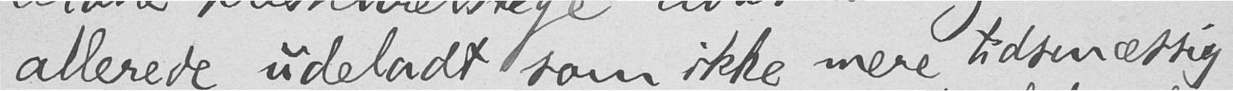allerede udeladt som ikke mere tidsmæssig
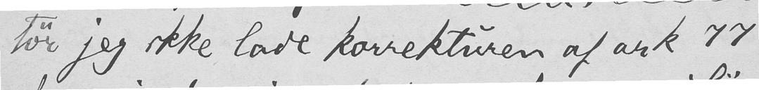tør jeg ikke lade korrekturen af ark 77
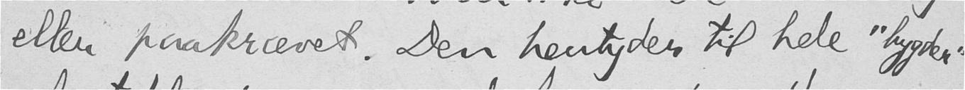eller paakrævet. Den hentyder til hele "bygder"
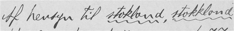Af hensyn til stokland, stokklond
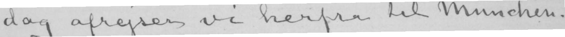dag afrejser vi herfra til München.
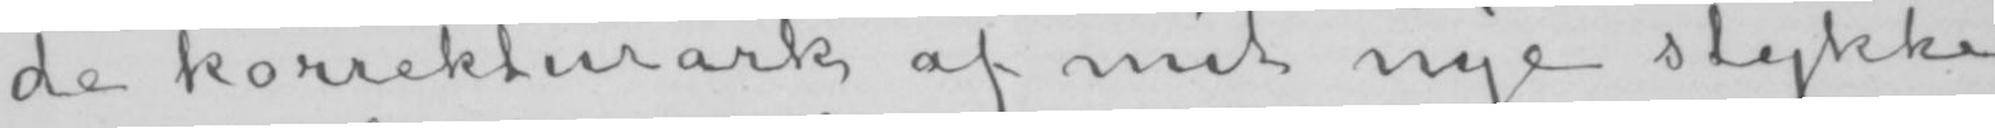de korrekturark af mit nye stykke
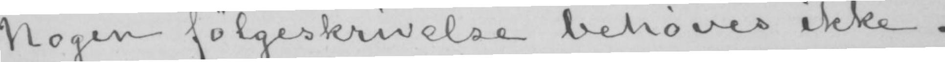Nogen følgeskrivelse behøves ikke.
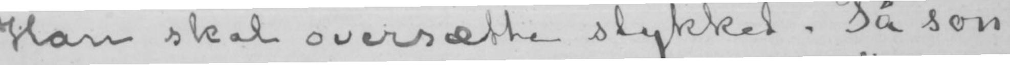Han skal oversætte stykket. På søn-
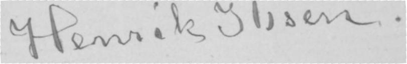Henrik Ibsen.
